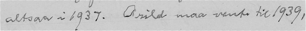altsaa i 1937. Arild maa vente til 1939,
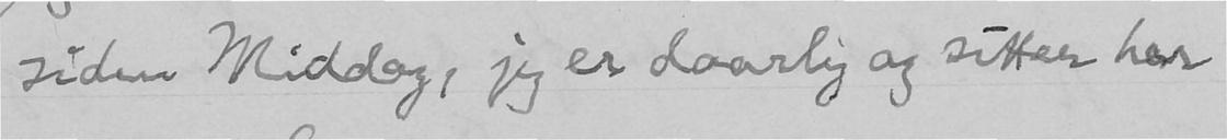siden Middag, jeg er daarlig og sitter her
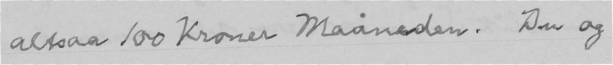altsaa 100 Kroner Maaneden. Du og
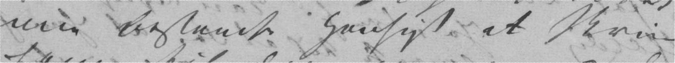min bestemte Hensigt at skrive
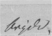bryder
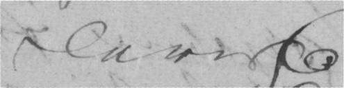Deres C.
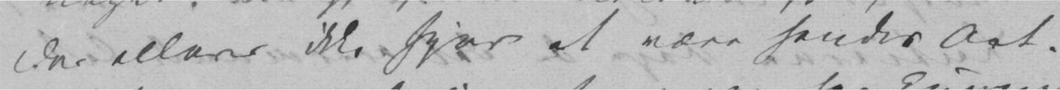der ellers ikke synes at være hendes Art.
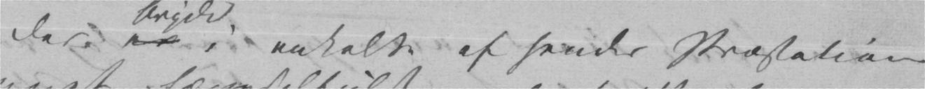Der er i enkelte af hendes Præstationer
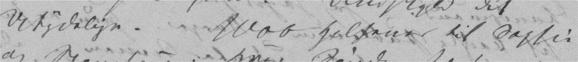Utydelige. 1000 Hilsener til Sophie
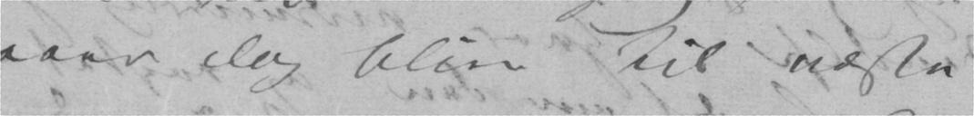faaer dog blive til næste
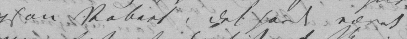ogsaa Robert, det havde været
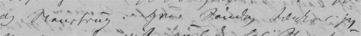og Stenstrup. Hver Søndag tænker jeg
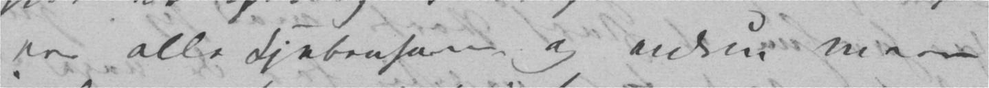er alle Kjøbenhavnere og endnu mere
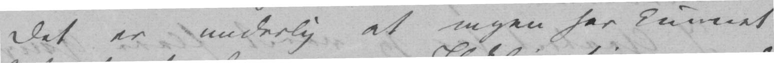Det er underlig at ingen har kunnet
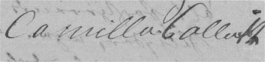Camilla Collett
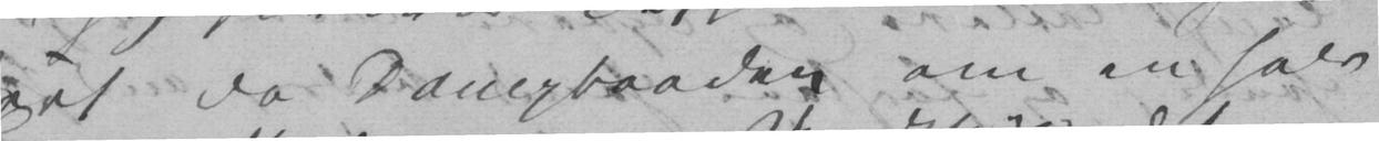Fart da Dampbaaden om en halv
